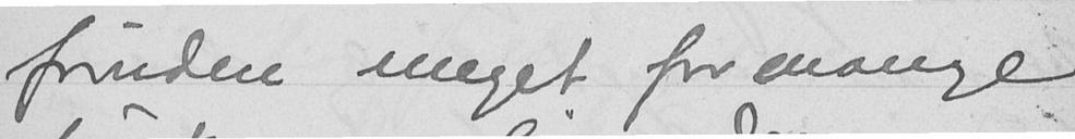foruden meget for mange
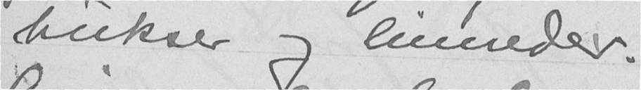bukser og linneder.
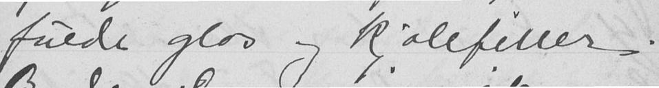fulde glas og kjolefiller.
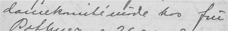dancekomitémøde hos fru
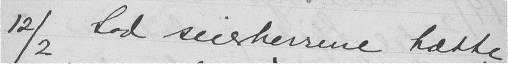12/2 Sad seierherrene tætte
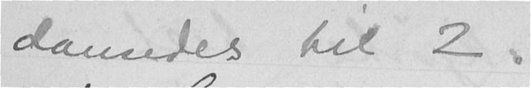dansedes til 2.
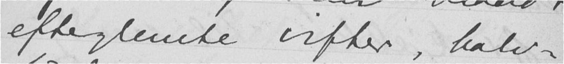efterglemte vifter, halv-
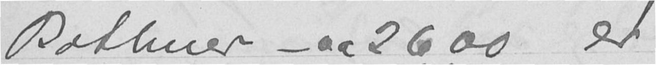Bothner - ca 2600 er
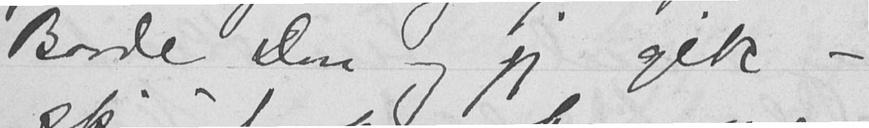Baade Don og jeg gik -
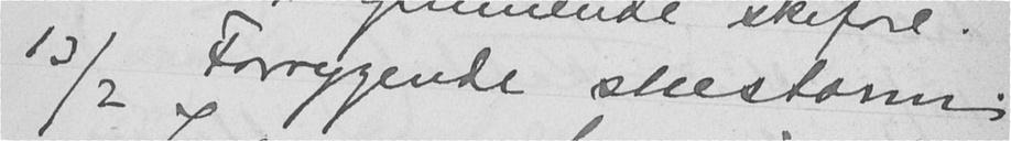13/2 Forrygende snestorm.
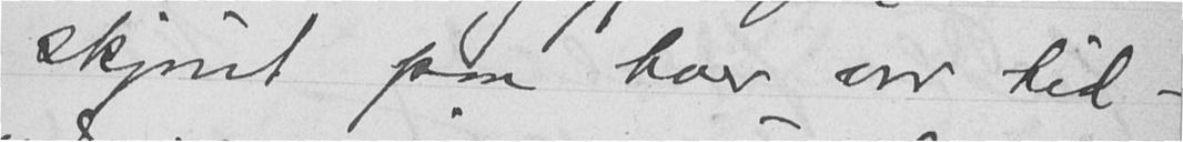skjønt paa hver vor tid -
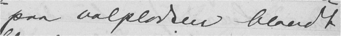paa valpladsen blandt
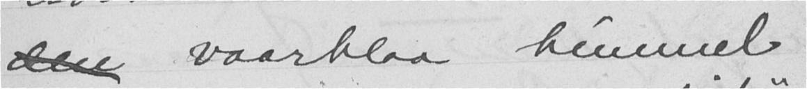den vaarblaa himmel
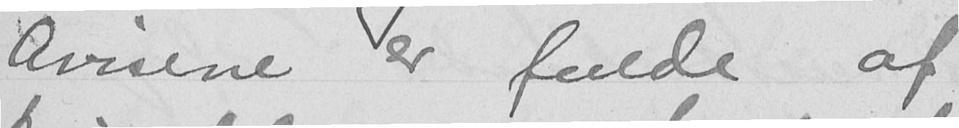Avisene er fulde af
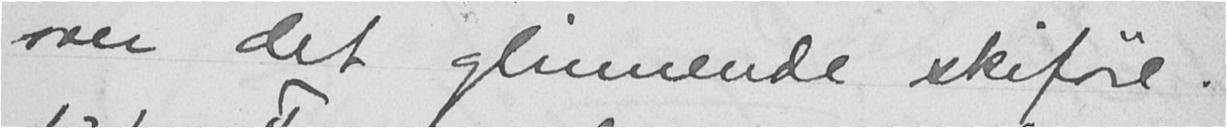over det glimrende skiføre.
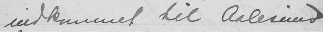indkommet til Aalesund
